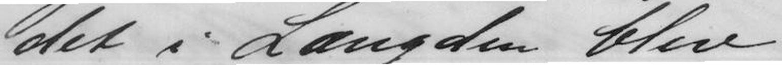det i Længden blev
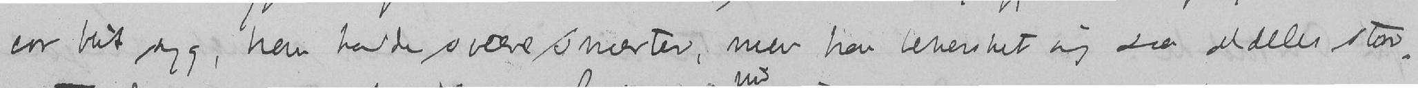var blit syg, han havde svære smerter, men han behersket sig saa aldeles stor
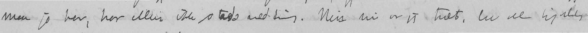maa jo her, har ellers ikke stads med ting. Nei nu er jeg træt, lev vel hjertelig
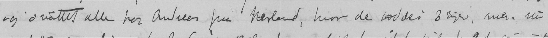og smittet alle hos Andreas paa Nerland, hvor de bodde i 3 uge, men nu
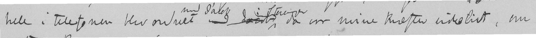hele i telefonen blev ordnet med Jacob i Stavanger, da var mine kræfter udslidt, om
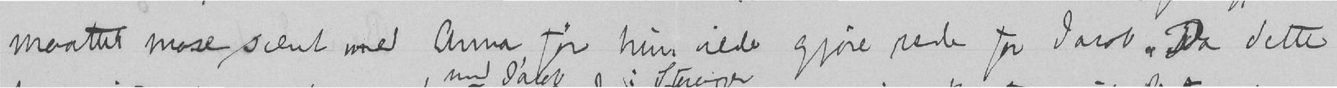maattet mase svært med Anna før hun vilde gjøre rede for Jacob. Da dette
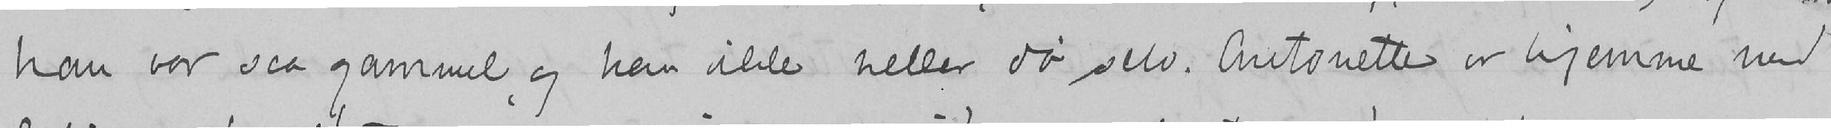han var saa gammel, og han vilde heller dø selv. Antonette er hjemme med
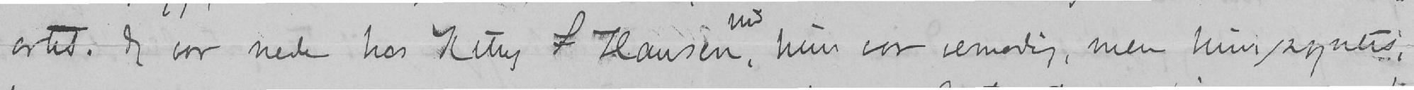artet. Jeg var nede hos Kitty S Hansen, hun var vemodig, men hun syntes,
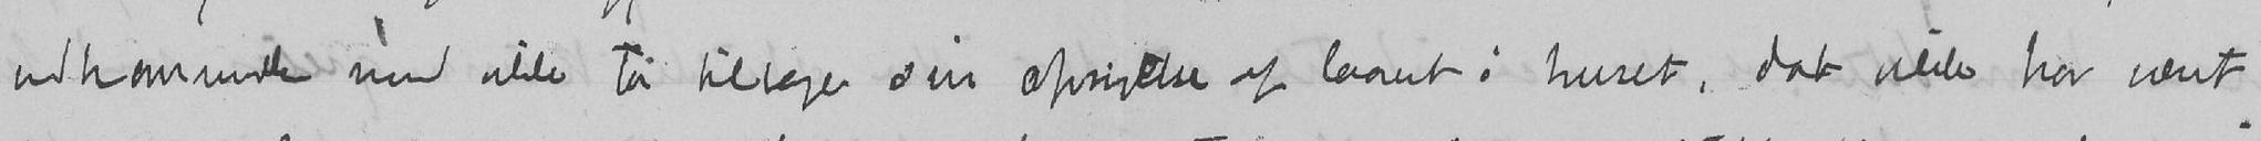vedkommende mon ville ta tilbage sin opsigelse af laanet i huset, det vilde ha vært
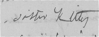søster Kitty
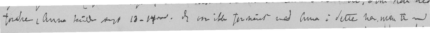fordre, Anna skulle havt . Jeg var ikke fornøiet med Anna i dette her, men ti
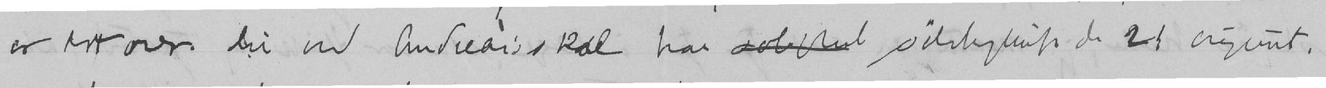er det over. Du ved Andreas skal paa sølvbrul sølvbryllup den 21 august,
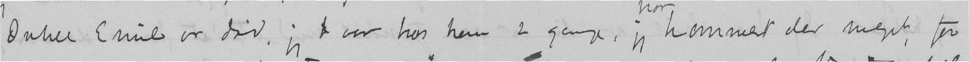Onkel Emil er død, jeg t var hos han 2 gange, jeg kommet der meget, for
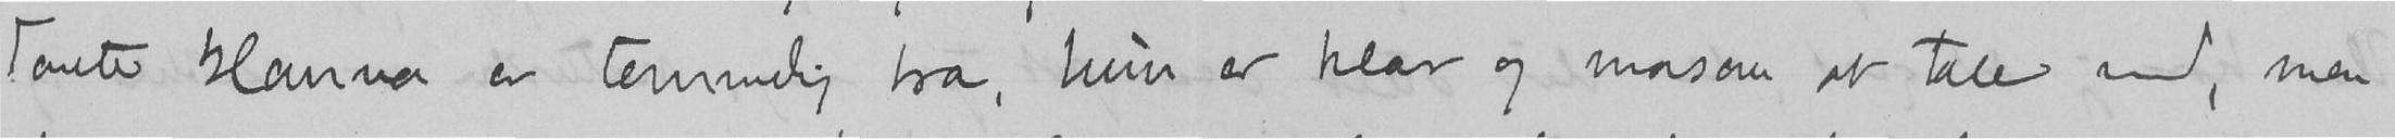Tante Hanna er temmelig bra, hun er klar og morsom at tale med, men
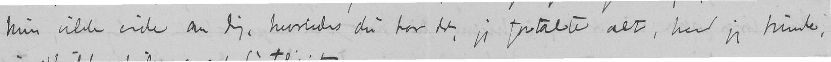hun vilde vide om dig, hvorledes du har det, jeg fortalte alt, hvad jeg kunde,
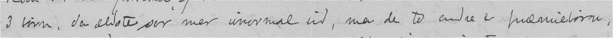3 børn, da ældste ser mer unormal ud, men de to andre er præmiebørn.
